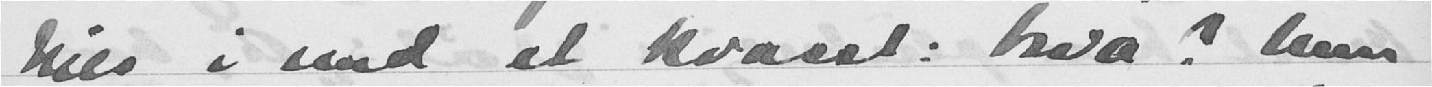Nils i med et kvasst; hva? Men
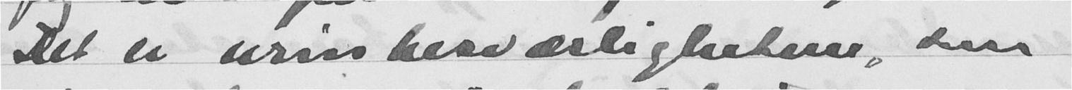Det er urinbesværlighetene, som
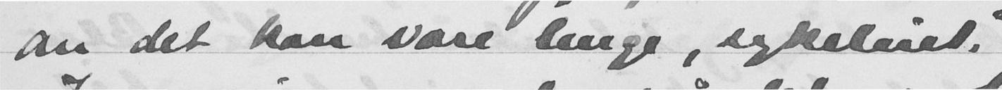om det kan vare lenge, sykeleiet.
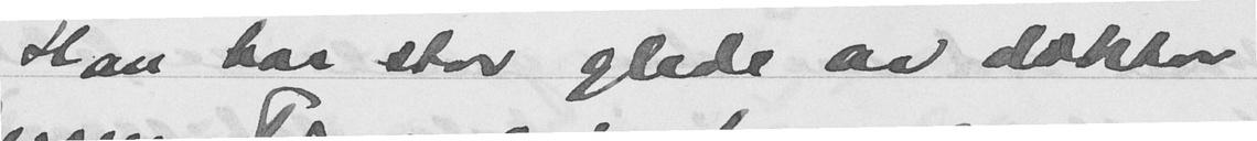Han har stor glede av doktor
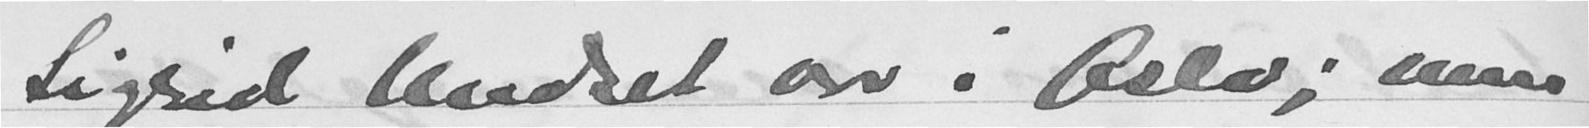Sigrid Undset var i Oslo; men
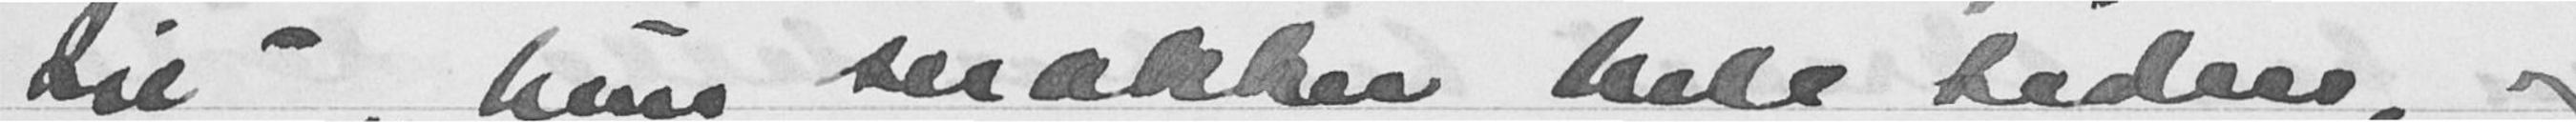lie", "hun snakker hele tiden, og
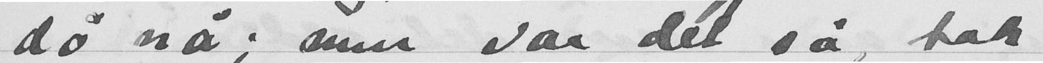dø nå; men var det så, tok
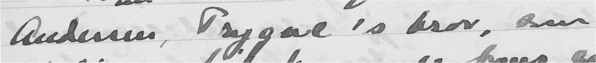Andersen, Trygve's bror, som
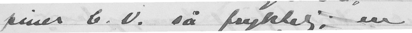piner K. V. så fryktelig, en
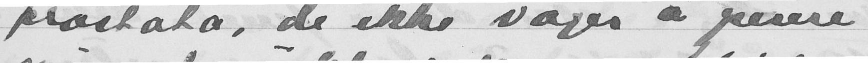prostata, de ikke våger å operere
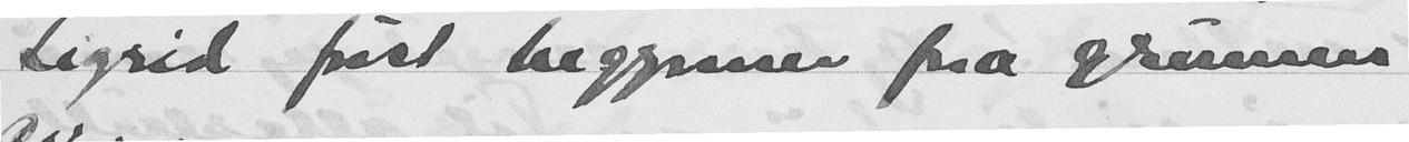Sigrid først begynner fra grunnen
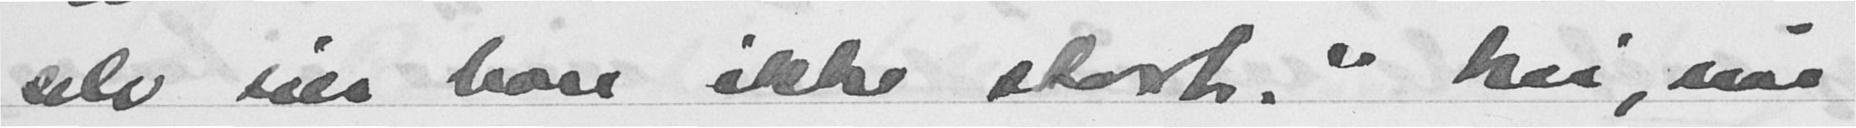selv sier han ikke stort." nei, når
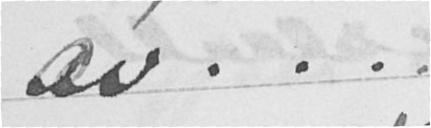av...
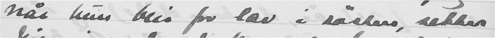når hun blir for lav i røsten, setter
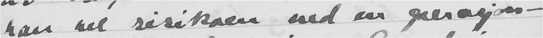han vel risikoen ved en operasjons-
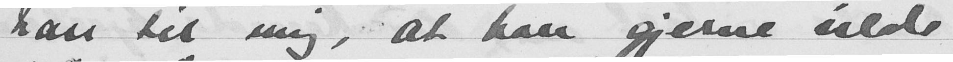han til mig, at han gjerne vilde
